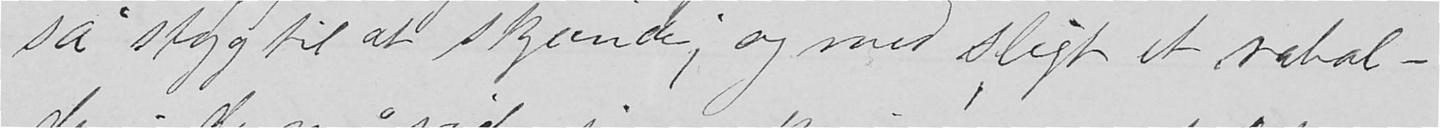så styg til at skjænde, og med sligt et rabal-
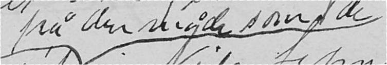på den måde som de
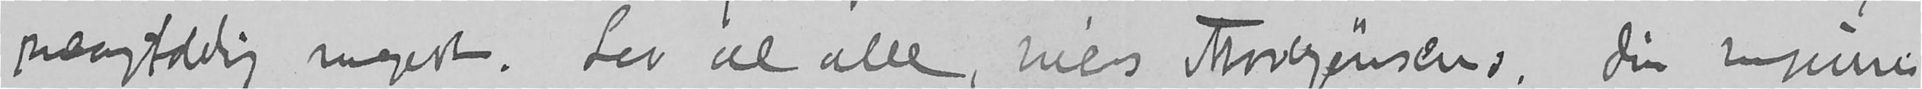mangfoldig meget. Lev vel alle, hils Thorgeirsens, din hengivne
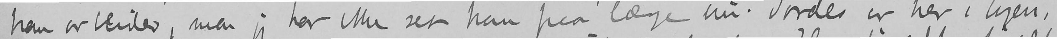han arbeider, men jeg har ikke set han paa længe nu. Jordes er her i byen,
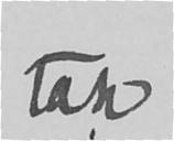tak
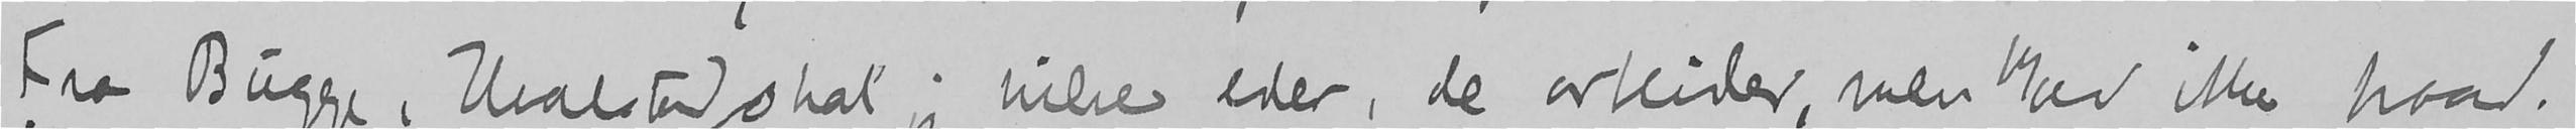Fra Bugge, Hvalstad skal jeg hilse eder, de arbeider, men jeg ved ikke hvad.
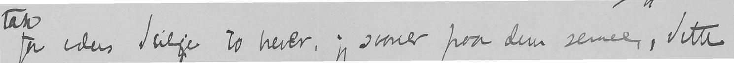for eders deilige to brever, jeg svarer paa dem senere, dette
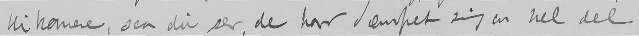blikanere, saa du ser, de har dæmpet sig en hel del.
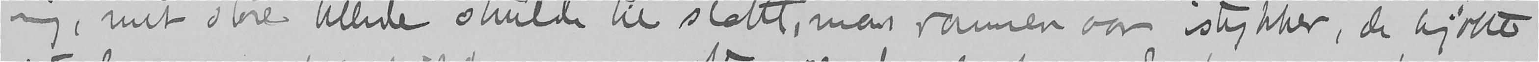mig, mit store billede skulde til slottet, men rammen var istykker, de kjøbte
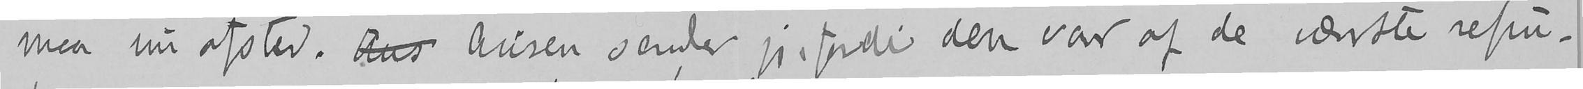maa nu afsted. Aus Avisen sender jeg, fordi den var af de værste repu-
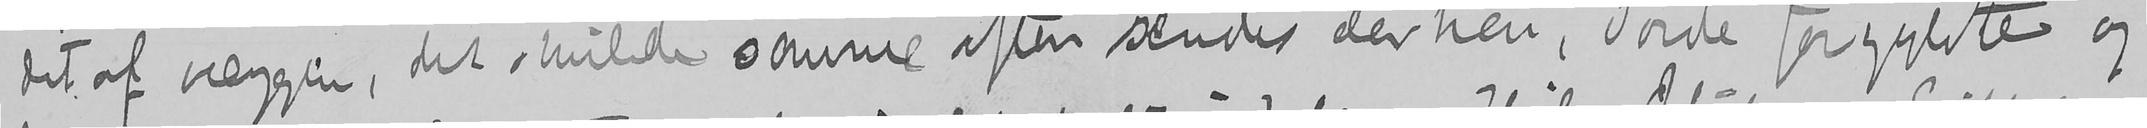det af væggen, det skulde samme aften sendes derhen, Jorde forgyldte og
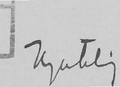Hjertelig
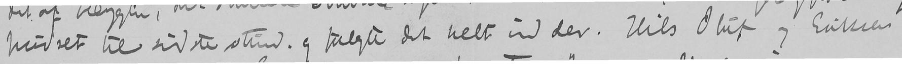pudset til sidste stund og fulgte det helt ind der. Hils Oluf og Eriksen
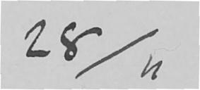28/11
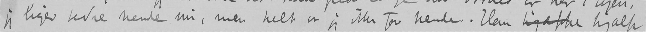jeg liger bedre hende nu, men helt er jeg ikke for hende. Han hjælpe hjalp
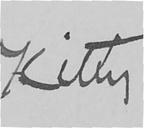Kitty
